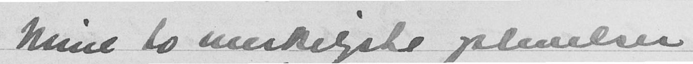Mine to mirkeligste plivelser
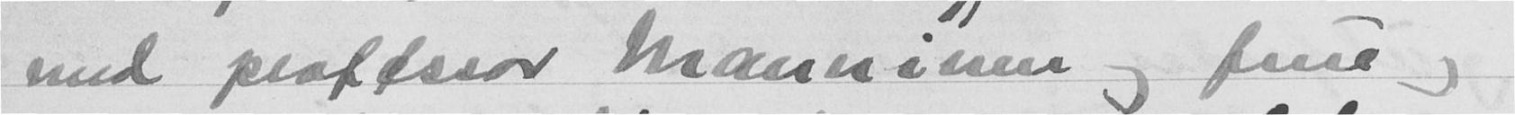med proftssor Maneisuen og fine og
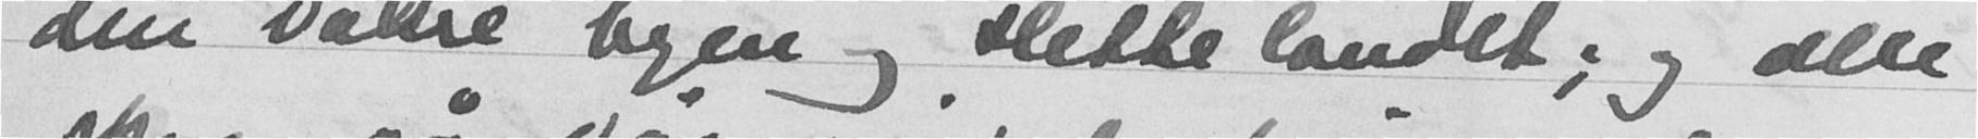den vakre byen og slette landet; og alle
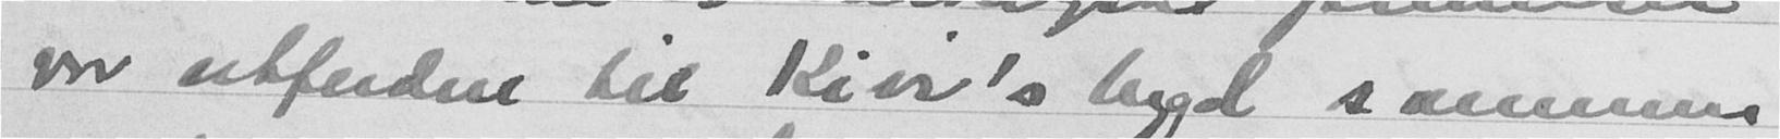var utferden til Kier's byd sammen
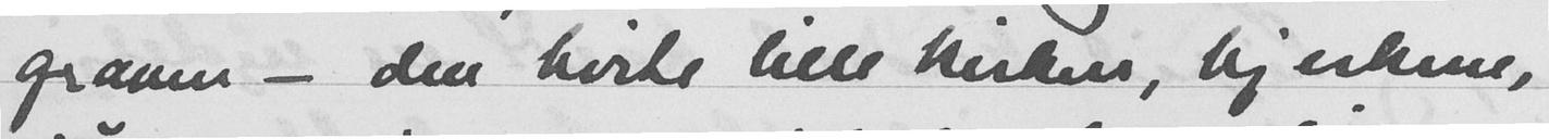graven - den hivste lille kiskers, hjerkene,
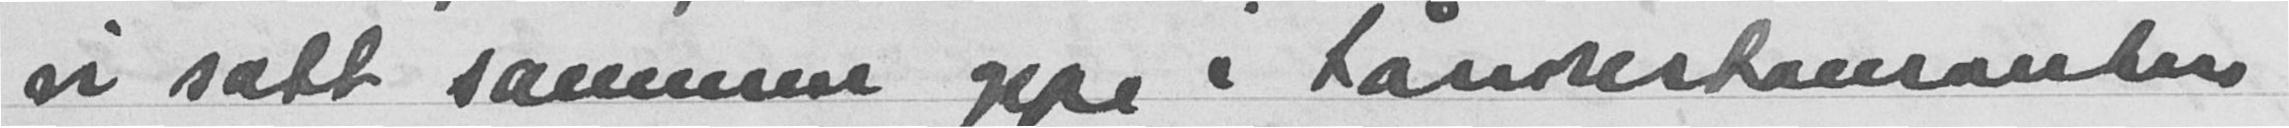vi satt sammen oppe i tårnrestauranten
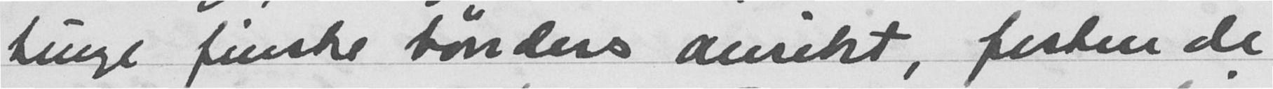tunge finske bønders ansikt, fisten de
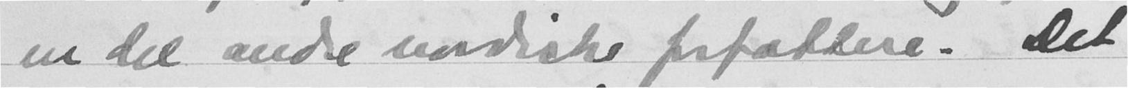en del andre nordiske forfattere. Det
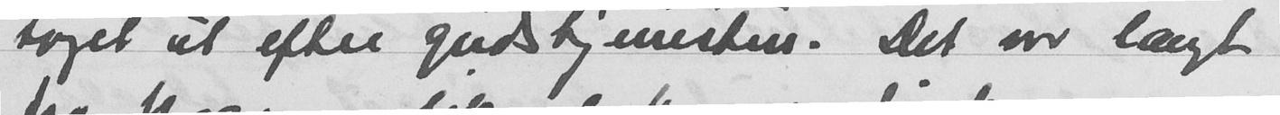toget ut efter gudstjensten. Det var langt
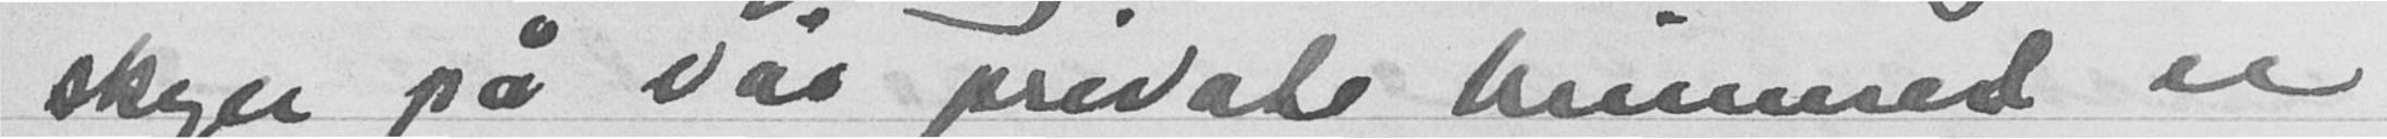skyer på var private himmet er
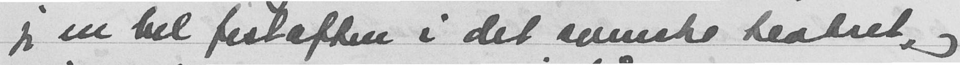jeg en hel festaften i det svenske teatret, og
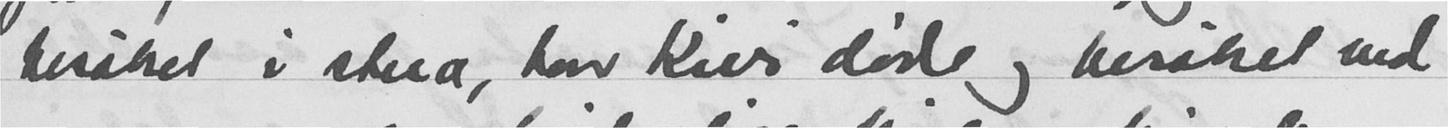besøkret i stua, hvor Kris døde og berøket ind
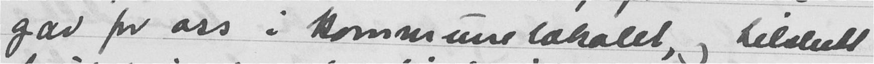gad for ors i kommunelokalet, og tilslutt
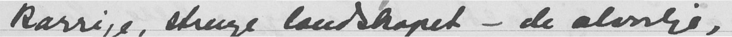Barrige, strenge landskapit - de alvorlige,
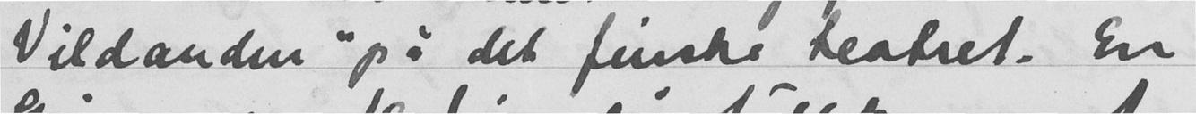Vildanden pi det finske teatret. En
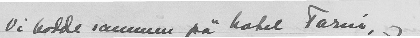Vi bodde sammen på hotel Parn, og
